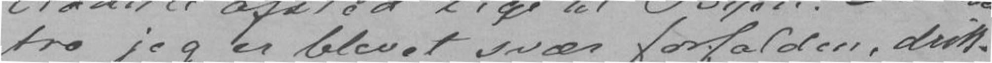tro jeg er blevet svær forfalden, drik-
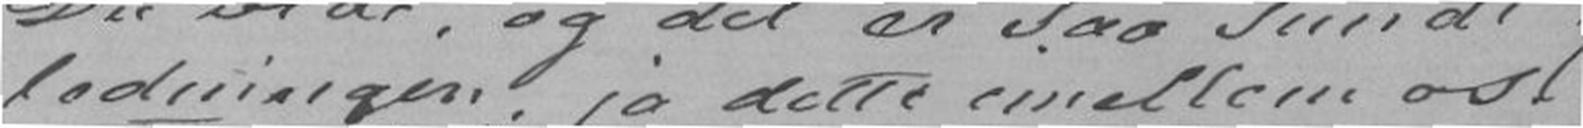ladninger, jo dette imellem os.
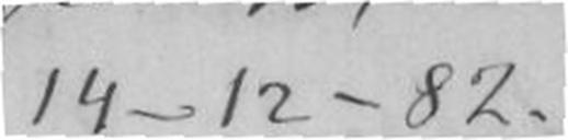14-12-82.
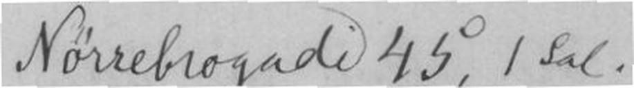Nørrebrogade 45, 1 Sal.
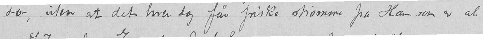dø, uten at det hver dag får friske strømme fra Ham som er al
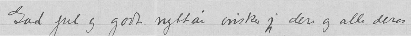God jul og godt nyttar ønsker jeg dere og alle deres
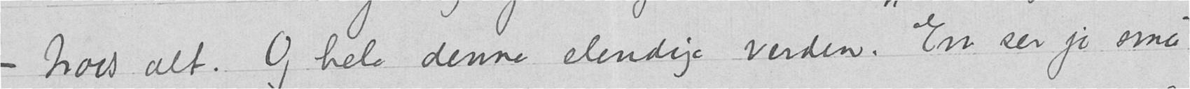– trods alt. Og hele denne elendige verden. En ser jo små
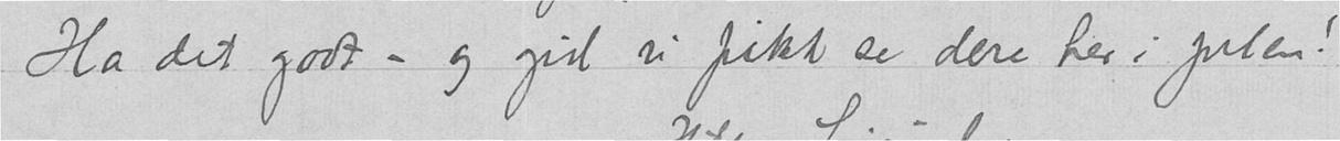Ha det godt – og gid vi fikk se dere her i julen!
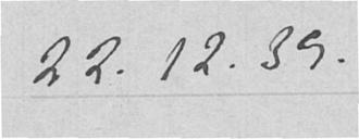22.12.39.
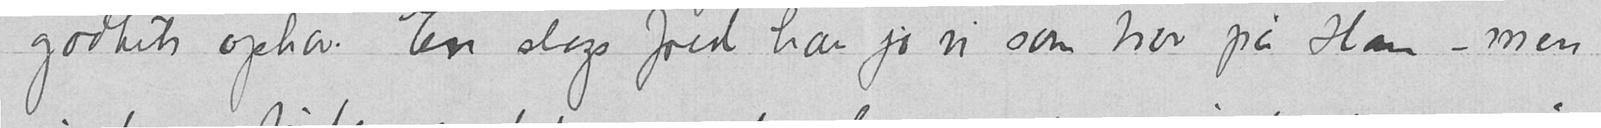godhets ophav. En slags fred har jo vi som tror på Ham – men
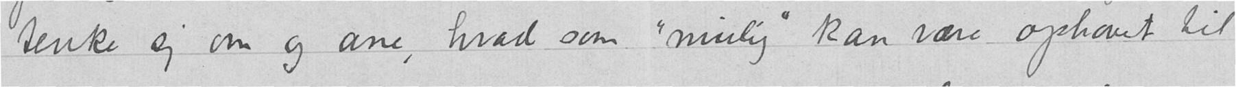tenke sig om og ane, hvad som "mulig" kan være ophavet til
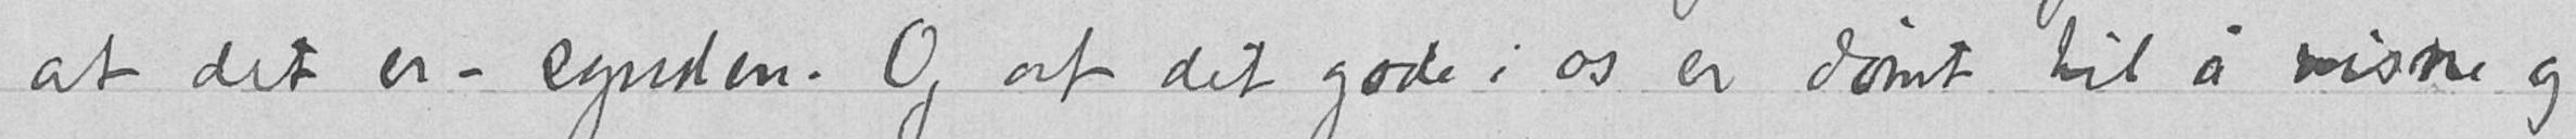at det er – synden. Og at det gode i os er dømt til å visne og
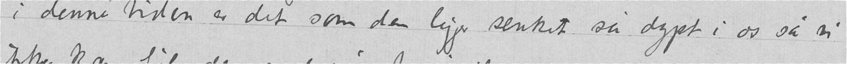i denne tiden er det som den ligger senket sa dypt i os så vi
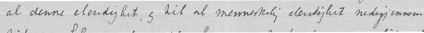al denne elendighet og til al menneskelig elendighet nedigjennem
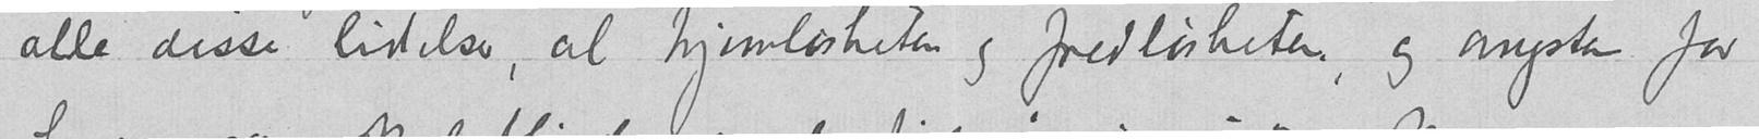alle disse lidelser, al hjemløsheten og fredløsheten, og angsten for
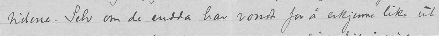tidene. Selv om de endda har vondt for å erkjenne like ut
